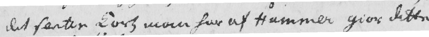det slette Kort man har af Hammer giør dette
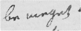be meget
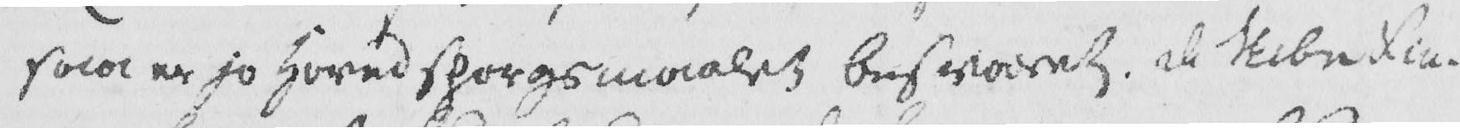saa er jo Hovedspørgsmaalet besvaret. de Skibe Fin-
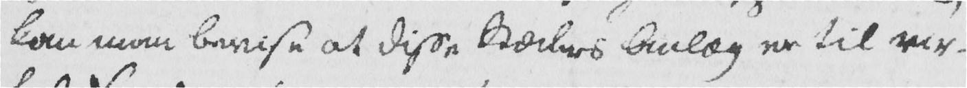kan man bevise at disse Stæders Anlæg er til vir-
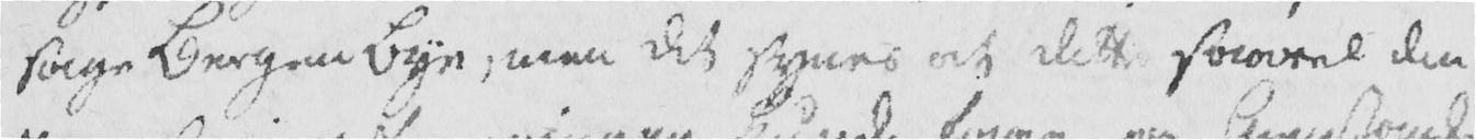sage Bergen Bye, men det synes at dette saavel den
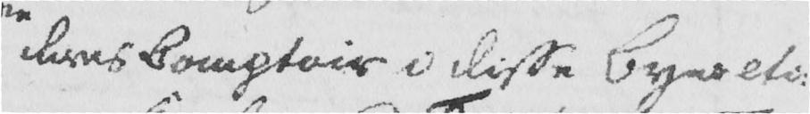deres Comptoir i disse Byer etc.
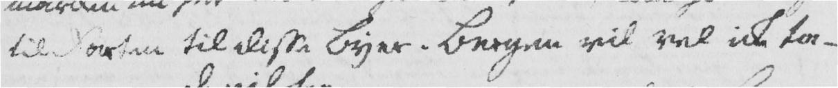til Farten til disse Byer. Bergen vil vel icke ta-
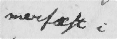merfæst i
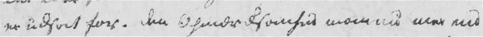er udsat for. den Opmærksomhed marud mer end
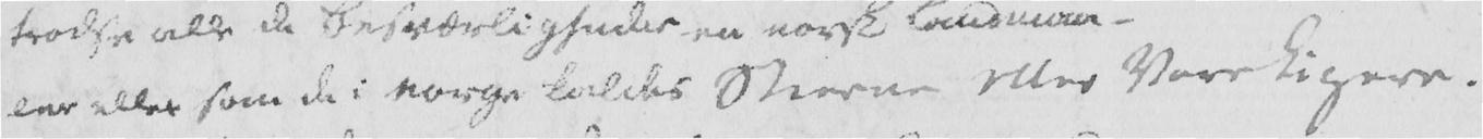ler eller som de i Norge kaldes Stierne eller Veir Kigere.
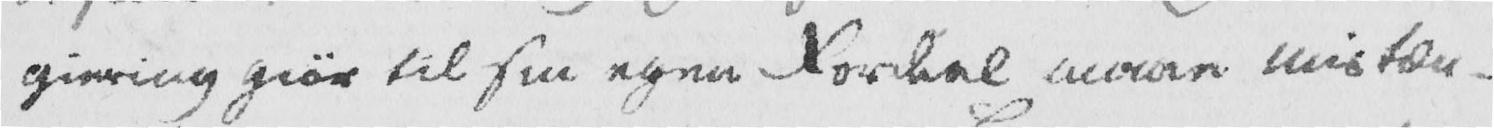giering giør til sin egen Fordeel maae mistæn-
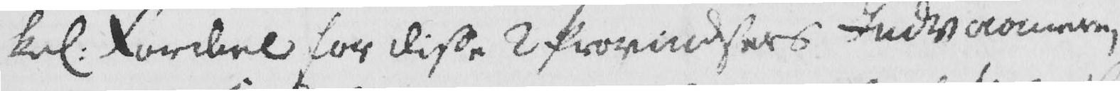kel. Fordeel for disse 2 Provindsers Indvaanere,
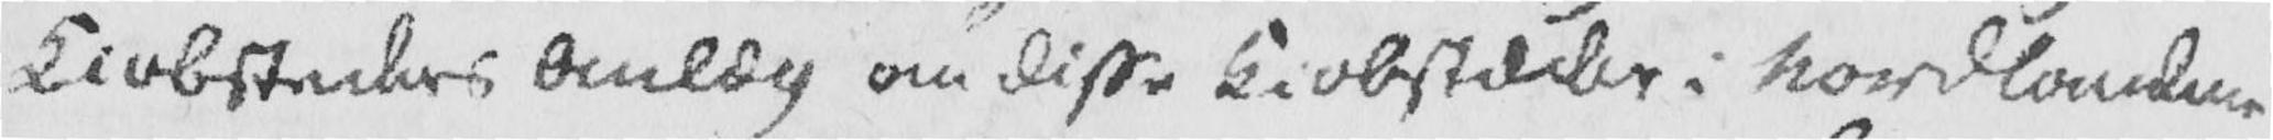Kiøbsteders Anlæg om disse Kiøbstæder i Nordlandene
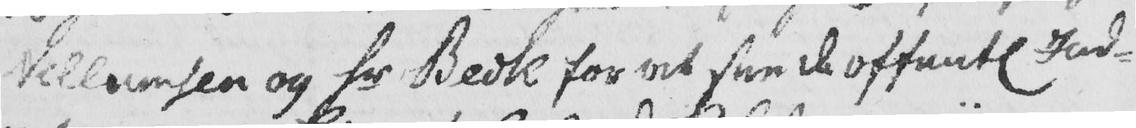Villumsen og Hr Beck for at see de offentl Ind-
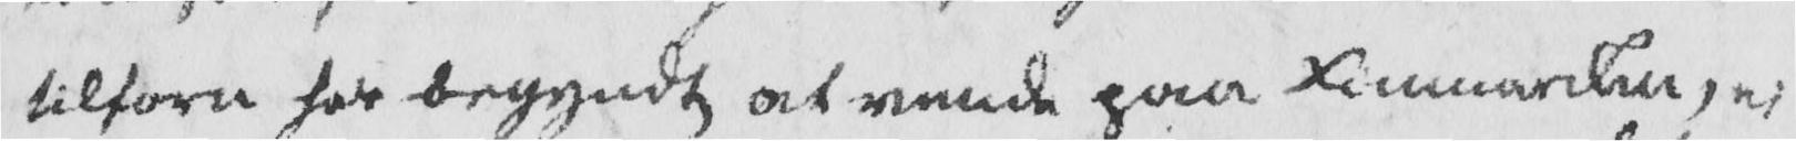tilforn har begyndt at vende paa Finmarken, at
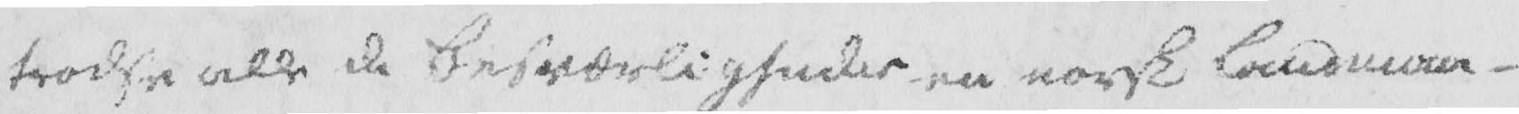trodse alle de Besværligheder en norsk Landmaa-
